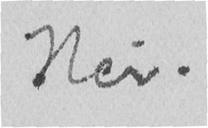Nei.
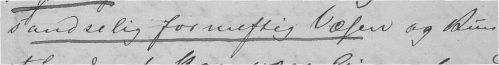sandselig fornuftig Væsen og kun
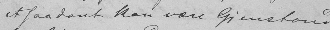et saadant kan være Gjenstand
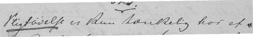Pligtøvelse er kun tænkelig hos et
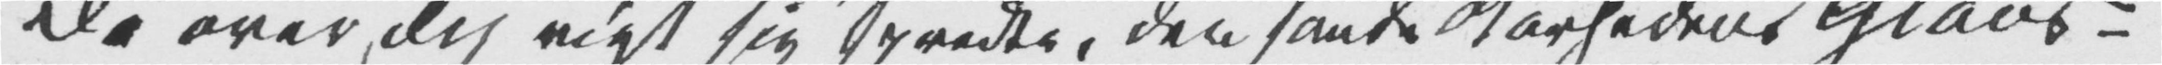Da over dig rigt sig spredte, den sande Storhedens Glans.
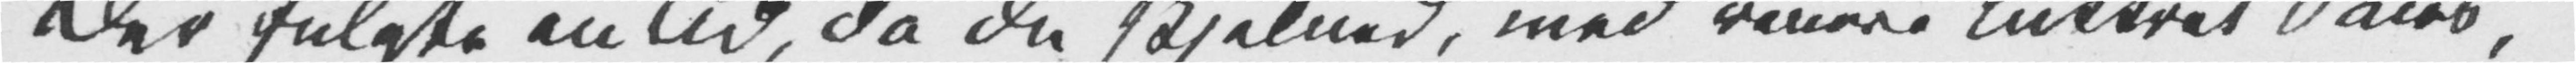Der fulgte en Tid, da du skjelned, med renere luttret Sans,
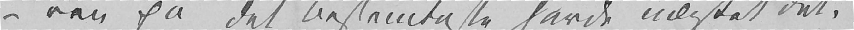ren på det bestemteste havde nægtet det.
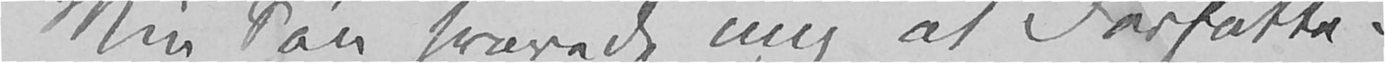Min Søn svarede mig at Forfatte¬
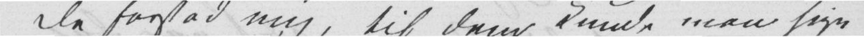De forstod mig, til dem kunde man sige
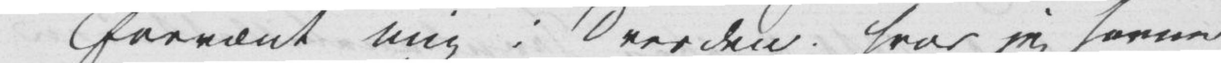forvænt mig i Dresden. Hvor jeg savne
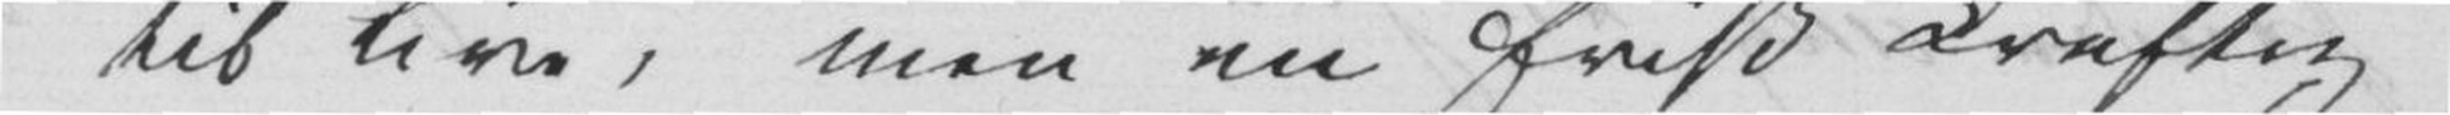til live, men en frisk kraftig
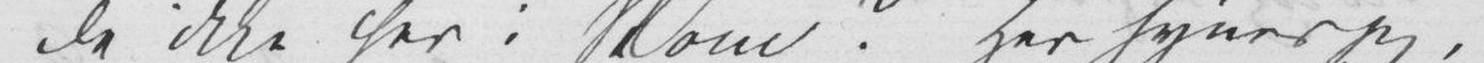De ikke her i Rom? Her synes jeg,
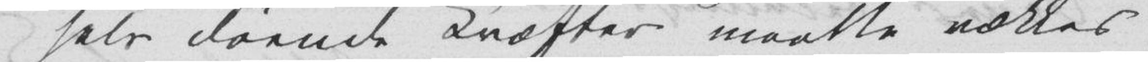selv døende Kræfter maatte vækkes
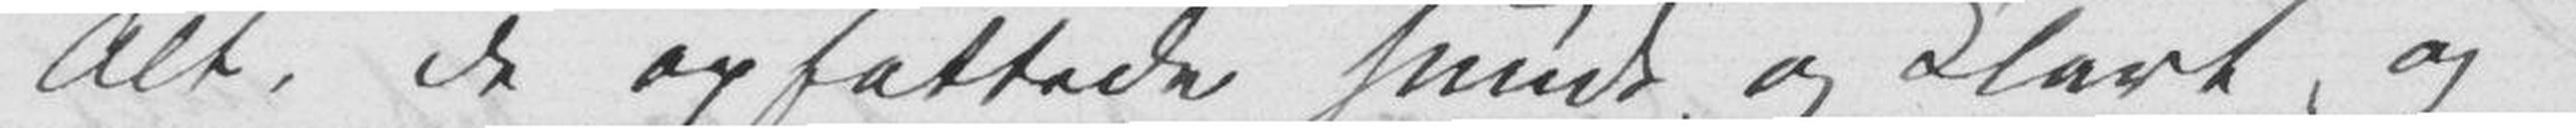Alt, de opfattede smuk og klart og
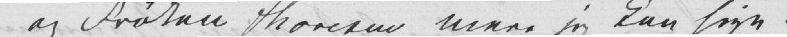og Frøken Thoresen mer jeg kan sige.
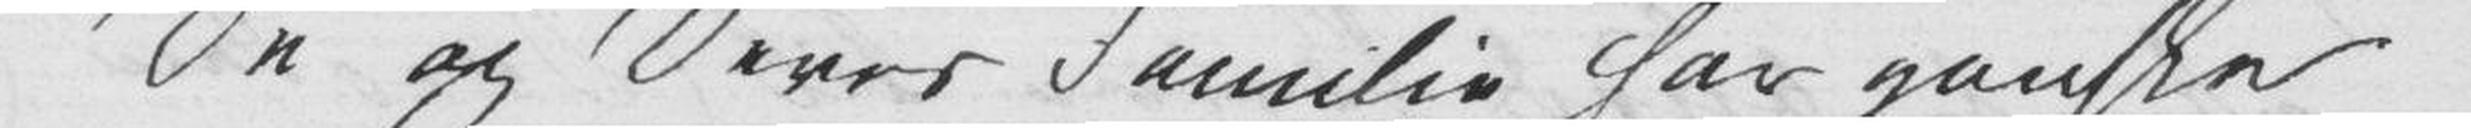Du og Deres Familie har ganske
